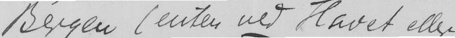Bergen (enten ved Havet eller
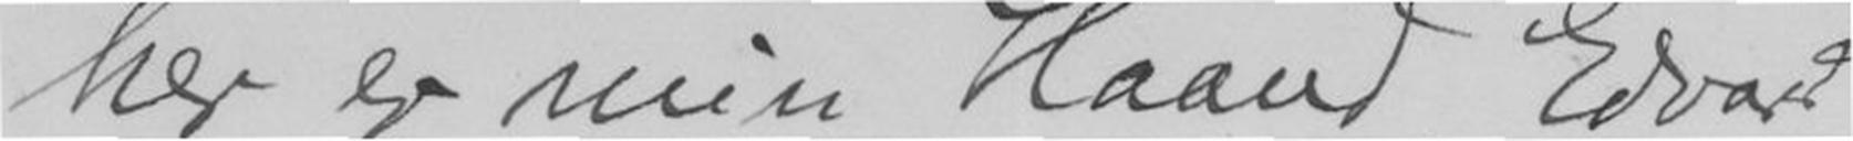her er min Haand Edvard
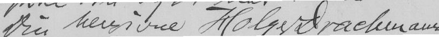Din hengivne Holger Drachmann
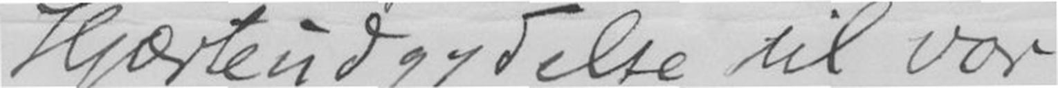Hjærteudgydelse til vor
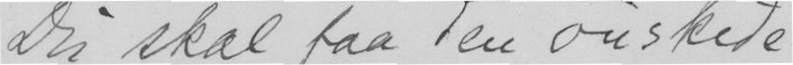Du skal faa den ønskede
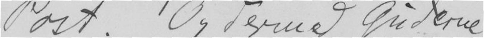Post. Og dermed Guderne
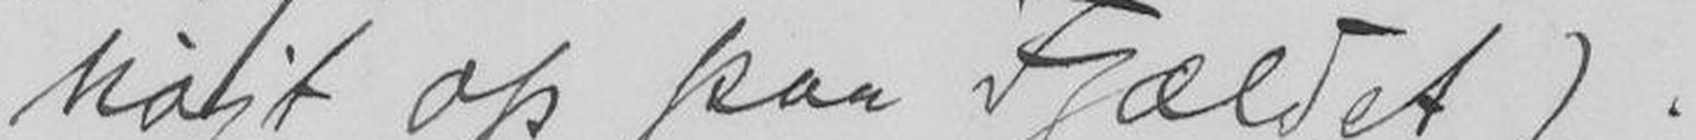højt op paa Fjældet).
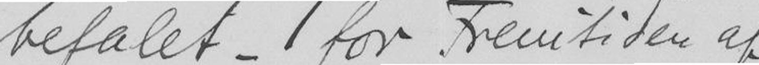befalet for Fremtiden af
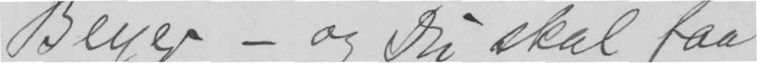Beyer - og Du skal faa
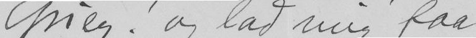Grieg! og lad mig faa
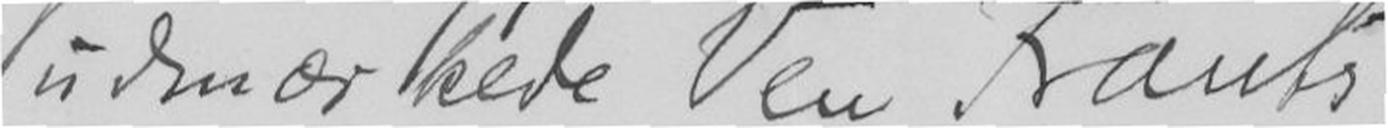udmærkede Ven Frants
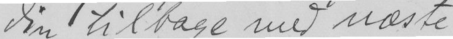din tilbage med næste
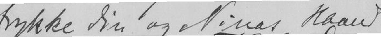trykke Din og Ninas Haand
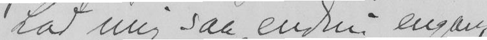Lad mig saa endnu engang
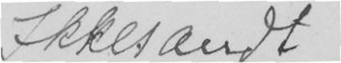Ikkesandt
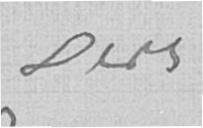Deres
